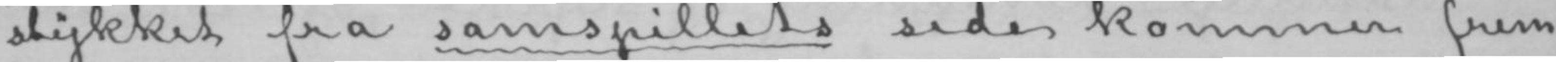stykket fra samspillets side kommer frem
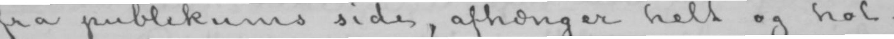fra publikums side, afhænger helt og hol-
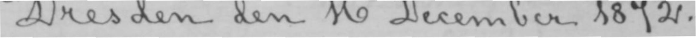Dresden den 16de December 1872.-
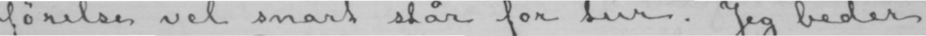førelse vel snart står for tur. Jeg beder
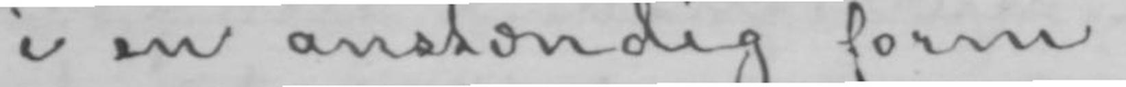i en anstændig form.
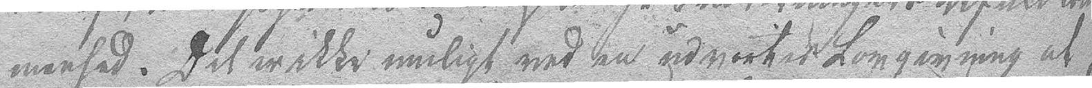menhed. Det ikke muligt ved en udvorter Lovgivning at
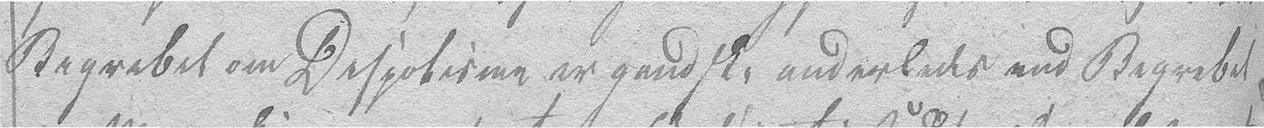Begrebet om Despotisme en gandske anderledes end Begrebet
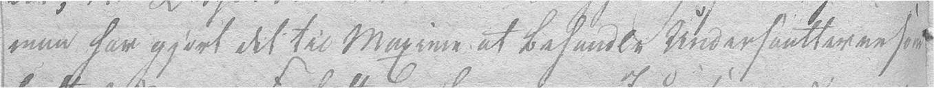man har gjort det til Maxime at behandle Undersaatterne som
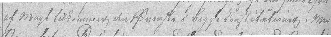af Magt tilkommer den Øverste i begge Konstitutioner. Men
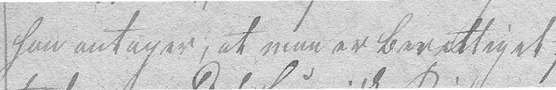han antager, at man er berettiget
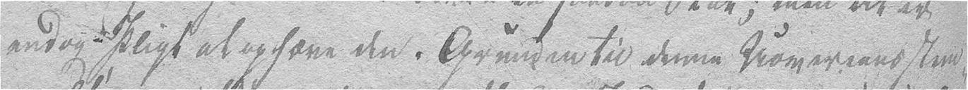endog Pligt at ophæve den. Grunden til denne Uovereensstem-
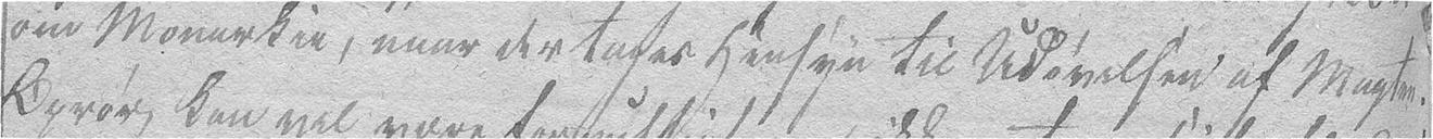om Monarkie, naar der tages Hensyn til Udøvelsen af Magten.
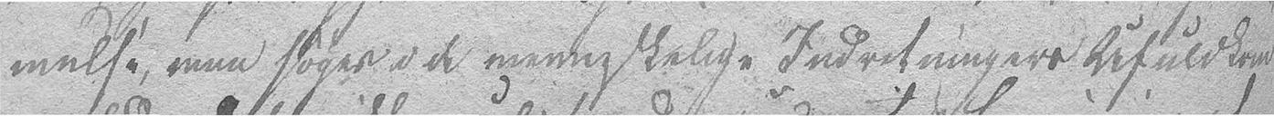melse, maa søges i de menneskelige Indretningers Ufuldkom-
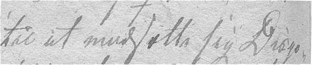til at modsætte sig Despo-
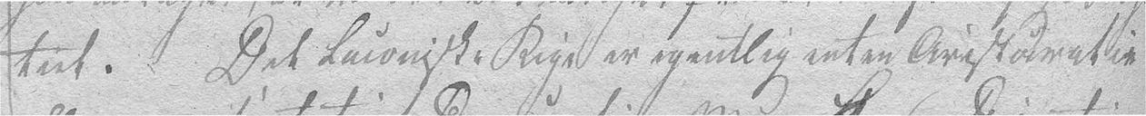tiet. Det Laconiske Rige er egentlig enten Aristocratie
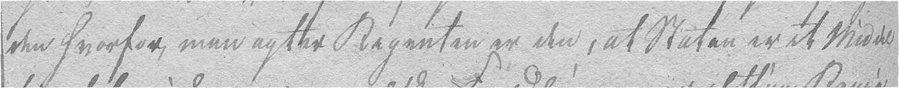den hvorfor man agter Regenten er den, at Staten er et Middel
